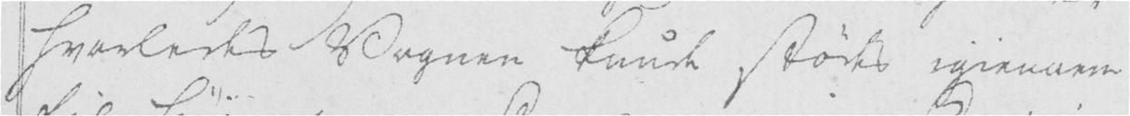hvorledes Vognen kunde stædes igiennem
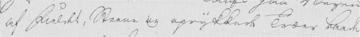af Fieldet, Steene og oprykkede Træer baade
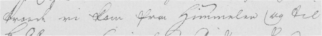troede vi kom fra Himmelen og til
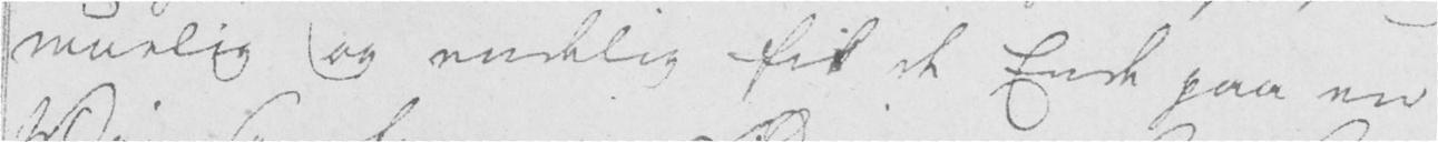muelig og endelig fik de Ende paa en
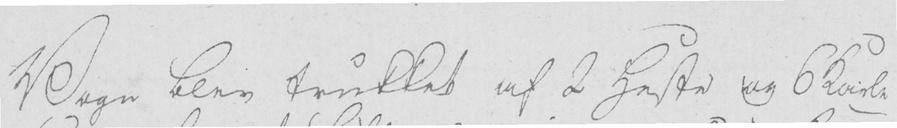Vogn blev trukket af 2 Heste og 6 Karle
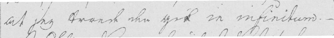at jeg troede den gik in infinitum. -
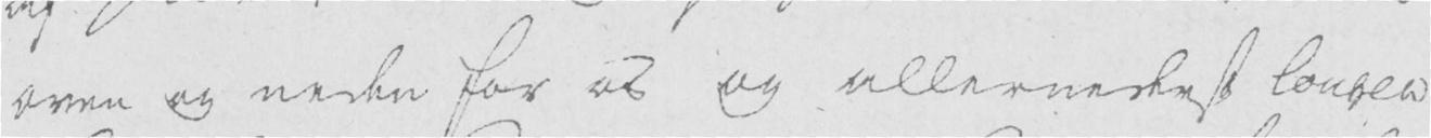oven og neden for os og allernederst longen
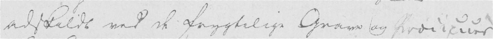adskildt ved de frygtelige Grave og Procipeer
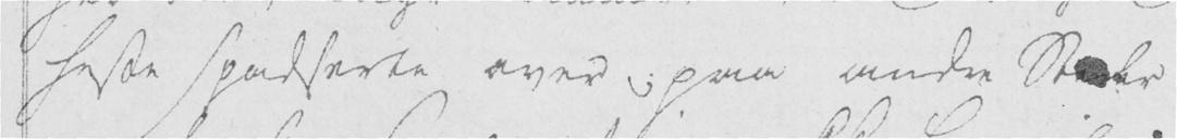heste spadserte over; paa andre Stæder
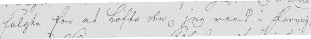fulgte for at løfte den; jeg reed i Forvey-
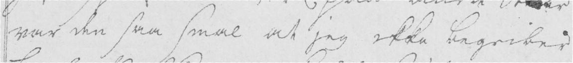var den saa smal at jeg ikke begriber
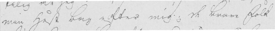min Hest bag efter mig; de brave Folk
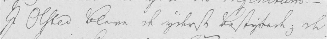I Olsted bleve de yderst bestyrtede; de
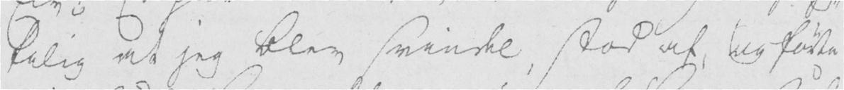telig at jeg blev svindel, stod af, og førte
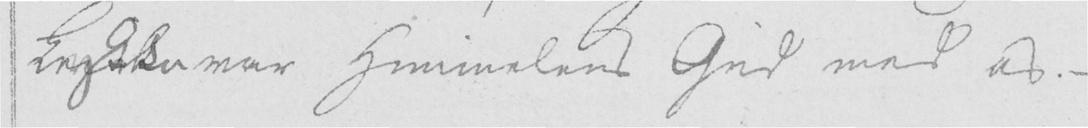Lykke var Himmelens Gud med os. -
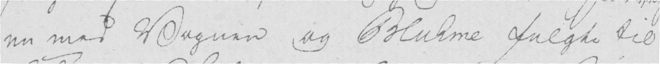en med Vognen og Bluhme fulgte til
